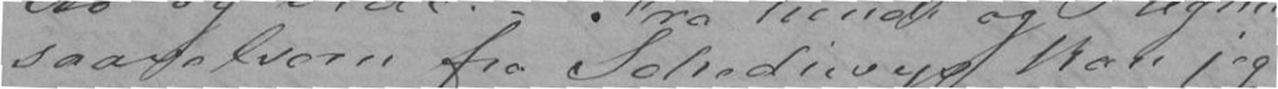saavelsom fra Schediwys kan jeg
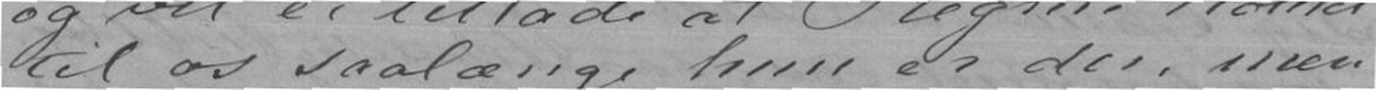til os saalenge hun er der, men
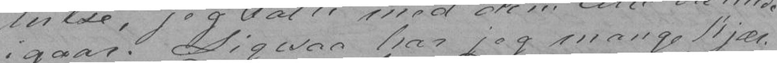igaar. Ligesaa har jeg mange kjær-
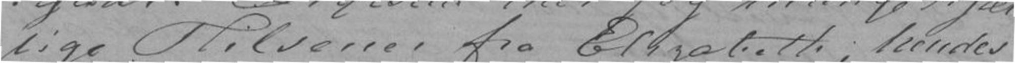lige Hilsener fra Elizabeth; hendes
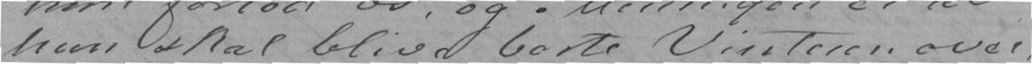hun skal blive borte Vinteren over,
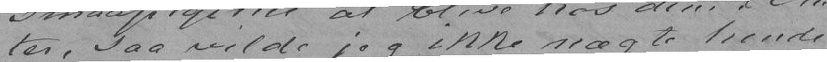ter, saa vilde jeg ikke nægte hende
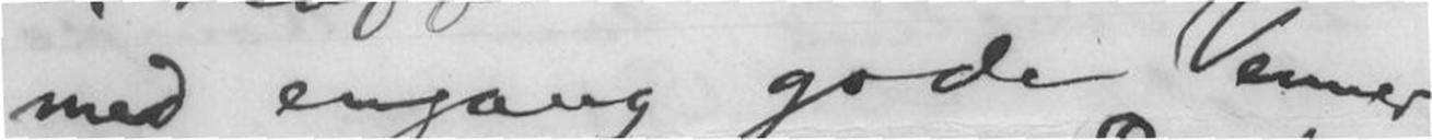med engang gode Venner.
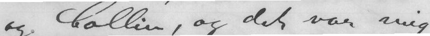og Collin, og det var mig
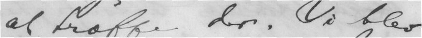at træffe der. Vi blev
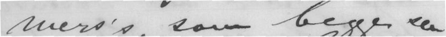mers's som begge sen-
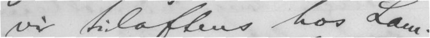vi tilaftens hos Lam-
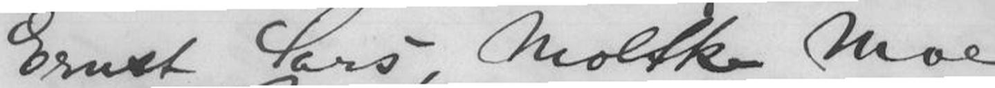Ernst Sars, Moltke Moe
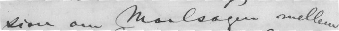sion om Maalsagen mellem
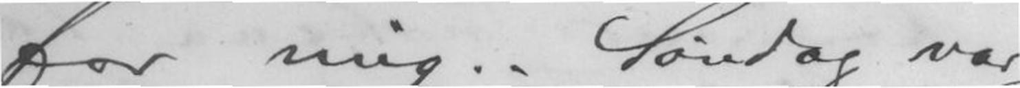for mig. Søndag var
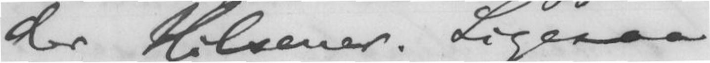der Hilsener. Ligesaa
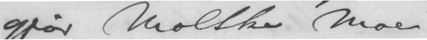gjør Moltke Moe,
